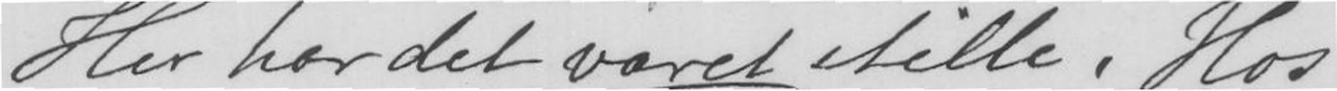Her har det været stille. Hos
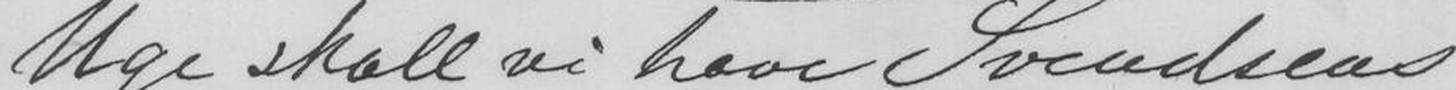Uge skall vi have Svendsens
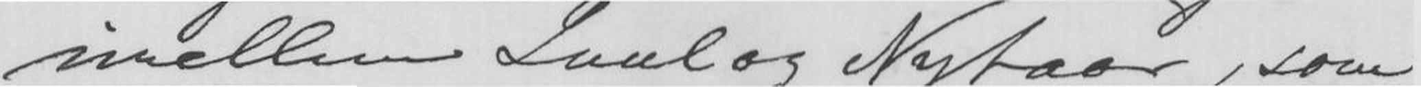mellem Juul og Nytaar, som
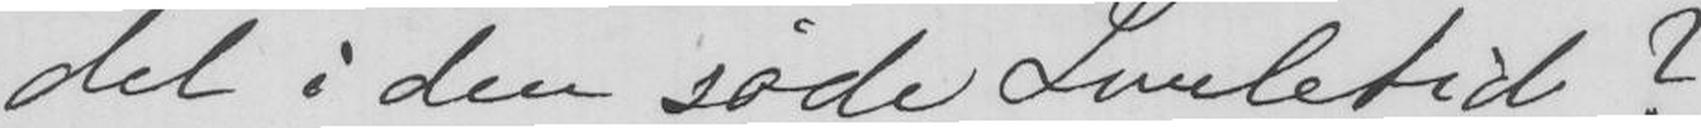det i den søde Juuletid?
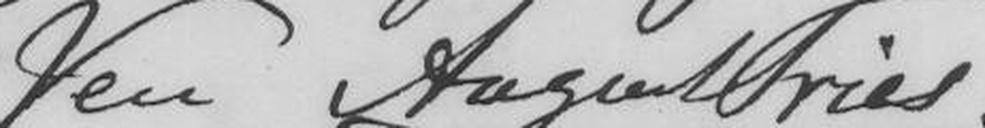Ven August Fries.
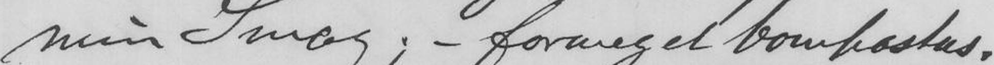min Smag; - formeget bombastus.
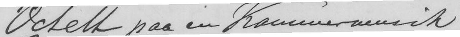Octett paa en Kammermusik
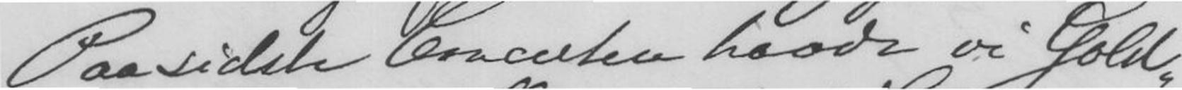Paa sidste Concerten havde vi Gold-
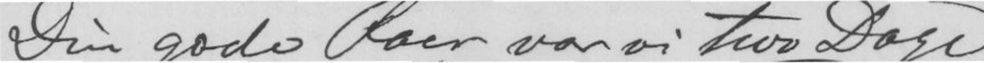Dim gode Faer var vi two Dage
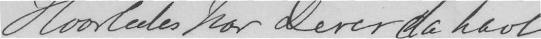Hvorledes har Derer da havt
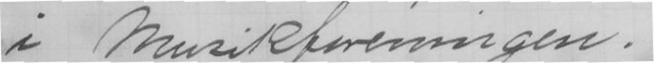i Musikforeningen.
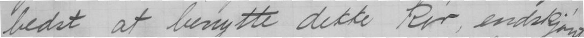bedst at benytte dette kor, endskjønt
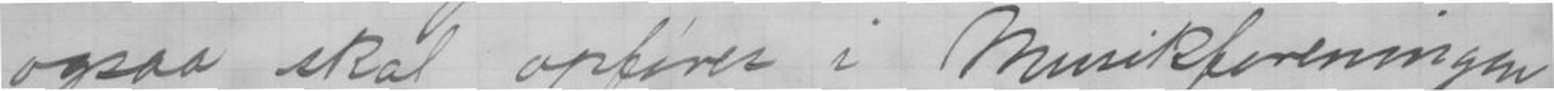ogsaa skal opføres i Musikforeningen
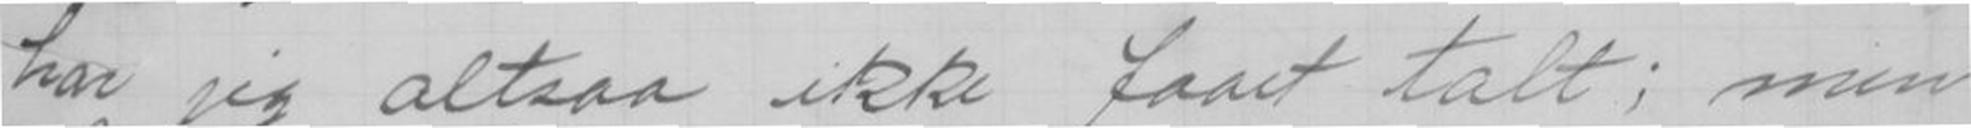har jeg altsaa ikke faaet talt; men
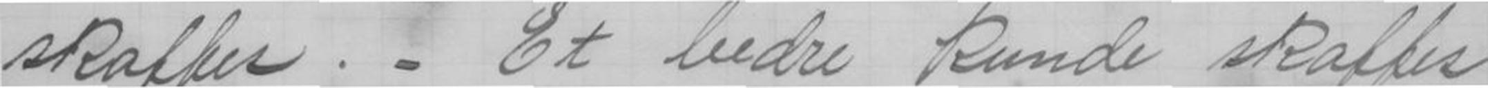skaffe. - Et bedre kunde skaffer
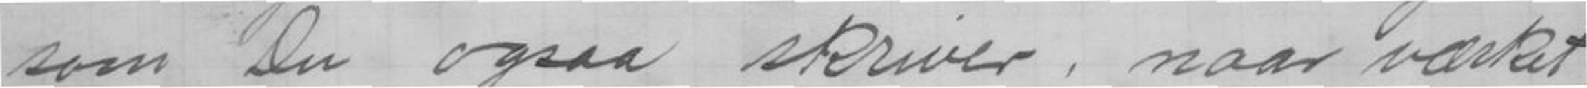som Du ogsaa skriver, naar værket
